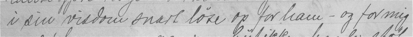i sin visdom snart løse op for ham - og for mig
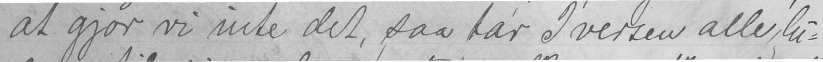at gjør vi inte det, saa tar Iversen alle lu-
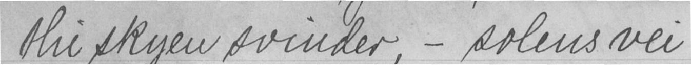thi skyen svinder, - solens vei
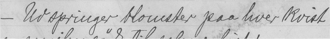- Ud springer blomster paa hver kvist.
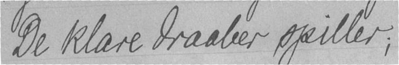De klare draaber spiller;
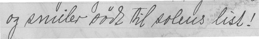og smiler sødt til solens list!
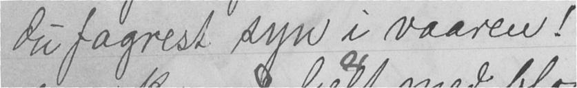du fagrest syn i vaaren!
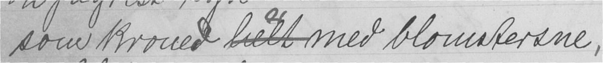som kroned helter med blomstersne,
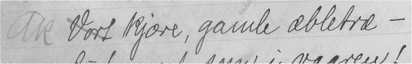Ak Vort kjære, gamle æbletræ -
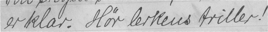er klar. Hør lerkens triller!
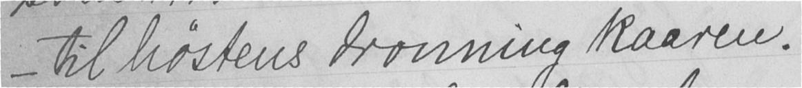- til høstens dronning kaaren.
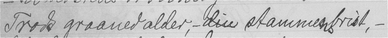Trods graaned alder, - din stammensbrist, -
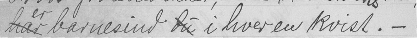harer barnesind du i hver en kvist. -
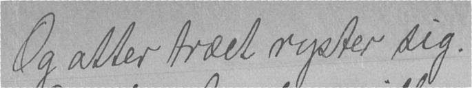Og atter træet ryster sig.
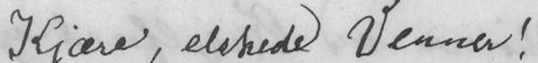Kjære, elskede Venner!
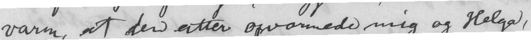varm, at den atter opvarmede mig og Helga,
